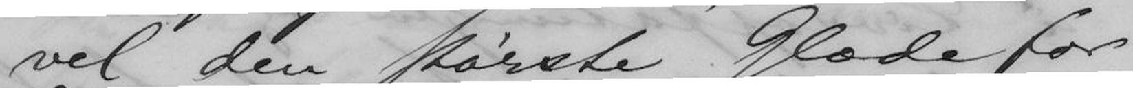vel den største Glæde for
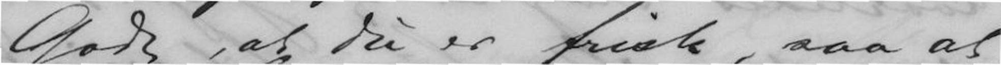Godt, at Du er frisk, saa at
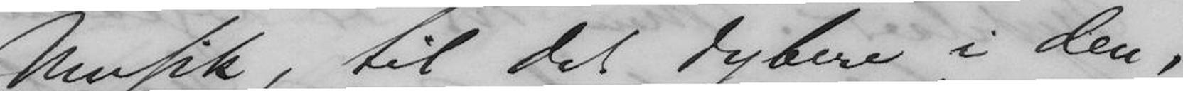Musik, til det dybere i den,
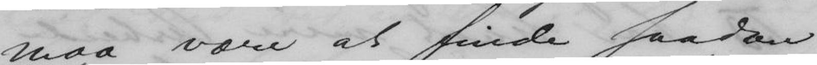maa være at finde saadan
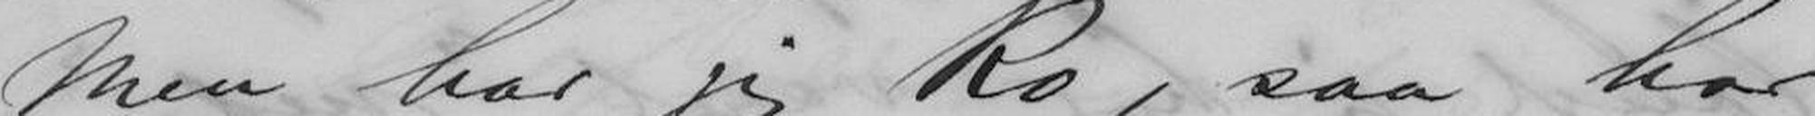Men har jeg Ro, saa har
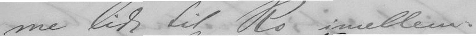me lidt til Ro imellem.
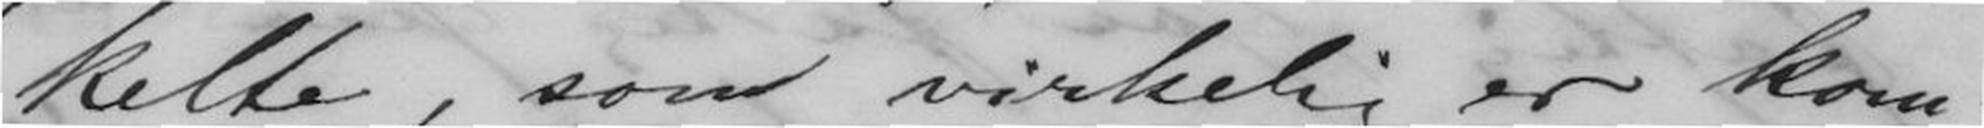kelte, som virkelig er kom-
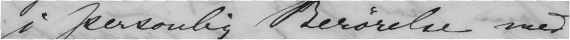i personlig Berørelse med
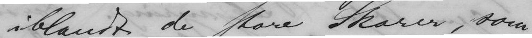iblandt de store Skarer, som
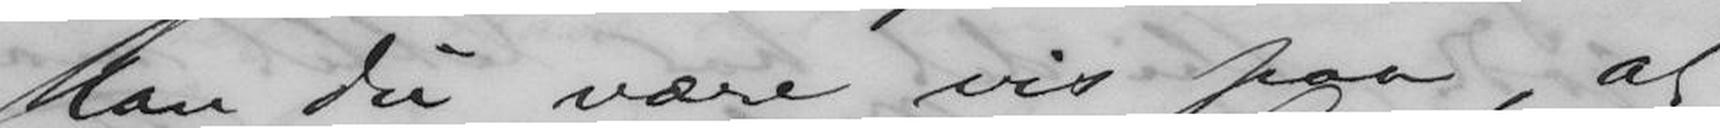kan Du være vis paa, at
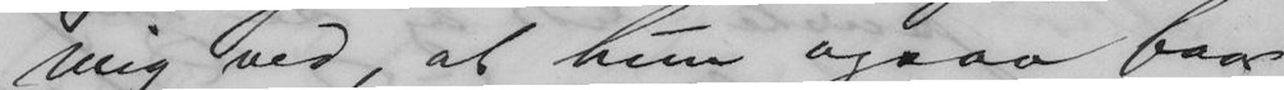mig ved, at hun ogsaa faar
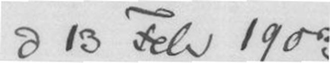d 13 Febr 1903
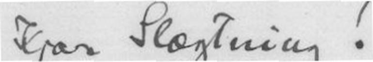Kjære Slægtning!
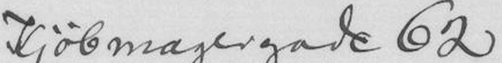Kjøbmagergade 62
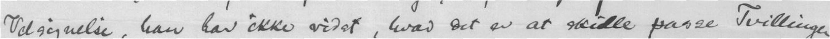Velsignelse, han har ikke vidst, hvad det er at skulle passe Tvillinger,
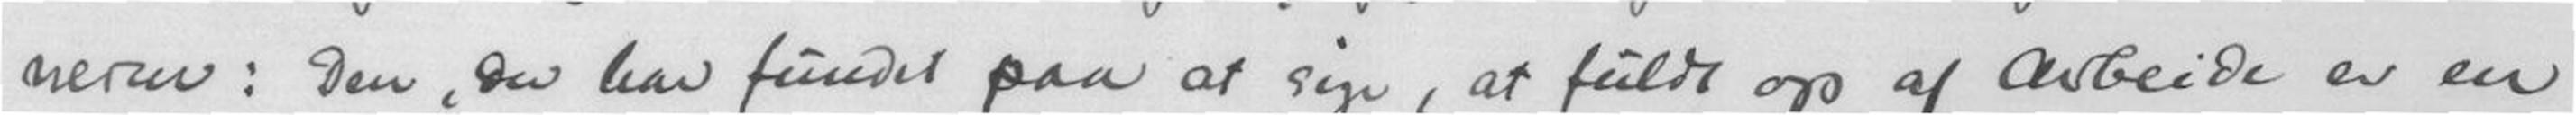nerver: Den, Du har fundet paa at sige, at fuldt op af Arbeide er en
